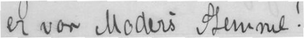er vor Moders Stemme!
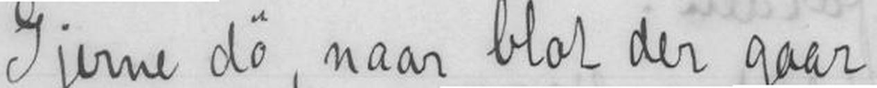Gjerne dø, naar blot der gaar
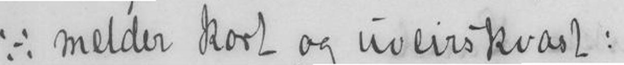:/: melder kort og uveirskvast:
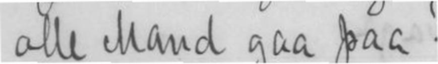alle Mand gaa paa!
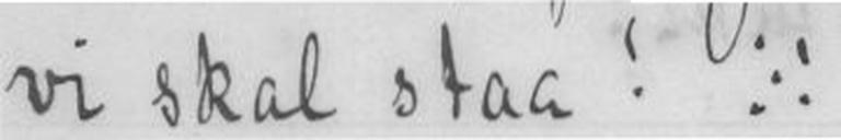vi skal staa! :/:
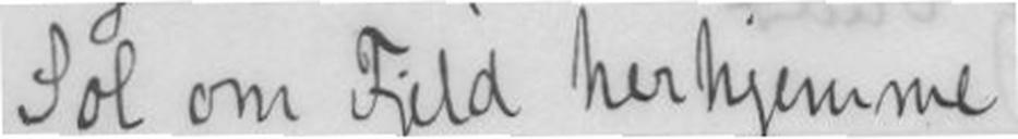Sol om Fjeld herhjemme
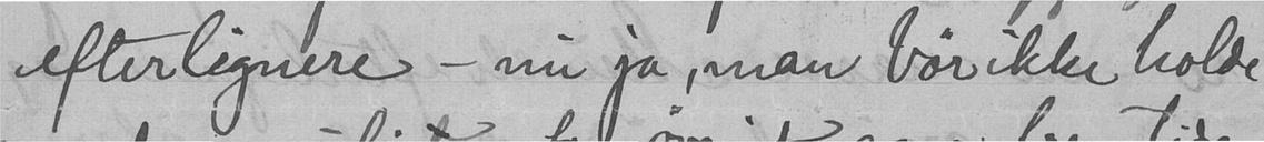efterlignere - nu ja, man bør ikke holde
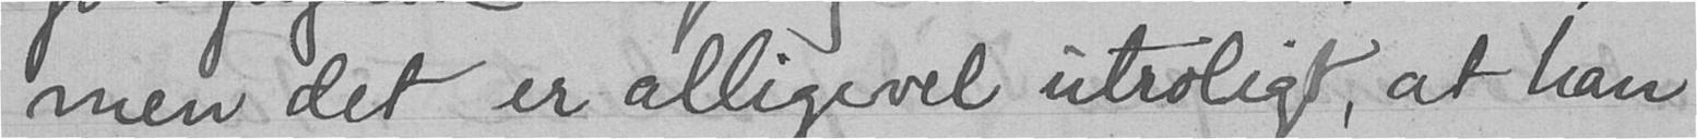men det er alligevel utroligt, at han
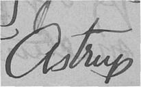Astrup
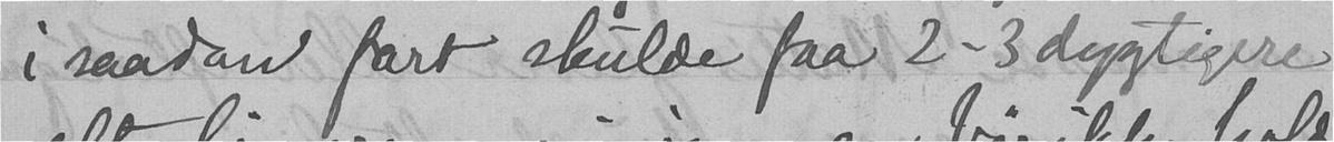i saadan fart skulde faa 2-3 dygtigere
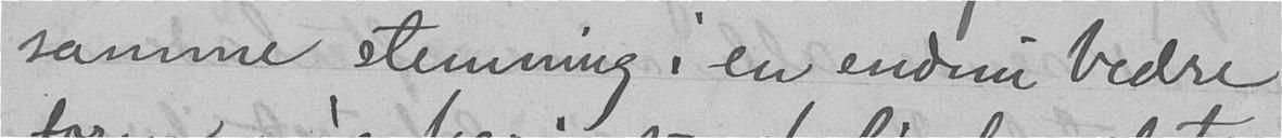samme stemning i en endnu bedre
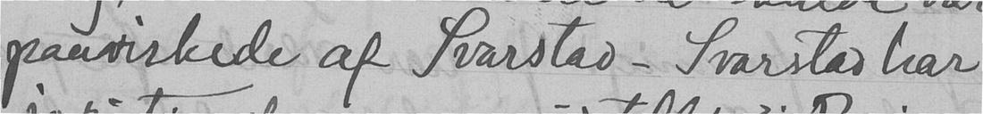paavirkede af Svarstad - Svarstad har
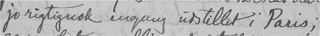jo rigtignok engang udstillet i Paris;
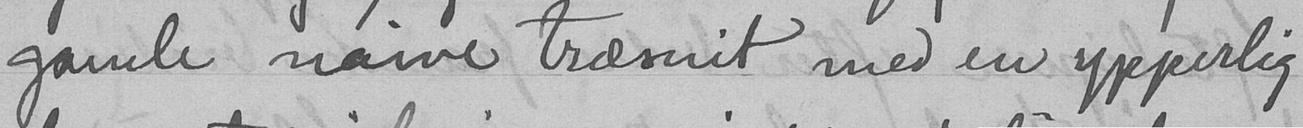gamle naive træsnit med en ypperlig
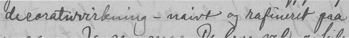decorativvirkning - naivt og rafineret paa
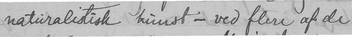naturalistisk kunst - ved flere af de
